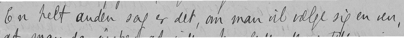En helt anden sag er det, om man vil vælge sig en ven,
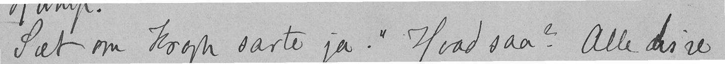Sæt om Krogh sarte ja." Hvad saa? Alle disse
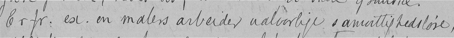Er fr. ex. en malers arbeider ualvorlige samvittighedsløse,
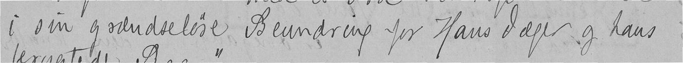i sin grændseløse Beundring for Hans Jæger og hans
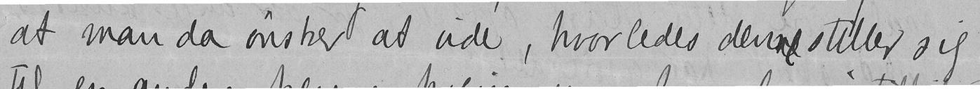at man da ønsker at vide, hvorledes denne stiller sig
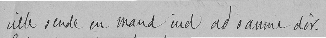vilde sende en mand ind at samme dør.
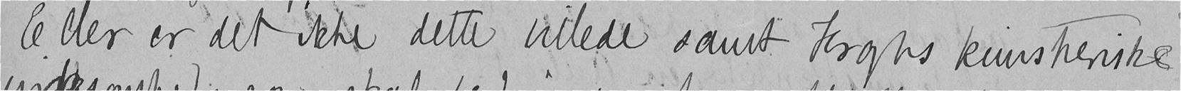Eller er det ikke dette billede samt Kroghs kunstneriske
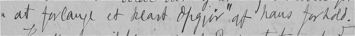"at forlange et klart Opgjør" af hans forhold.
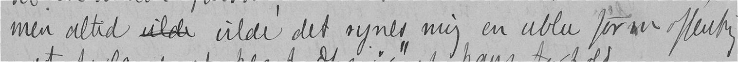men altid vilde vilde det synes mig en ublu form offentlig
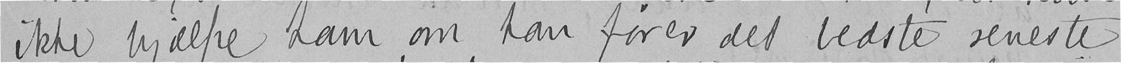ikke hjælpe ham, om han fører det bedste reneste,
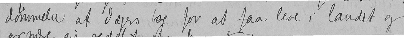dømmelse af Jægers bog for at faa leve i landet og
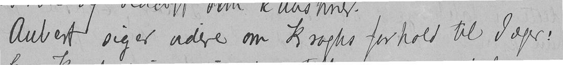Aubert siger videre om Kroghs forhold til Jæger,
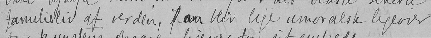familieliv af verden, han blir lige umoralsk ligeover
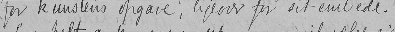for kunstens opgave, ligeover for sit embede.
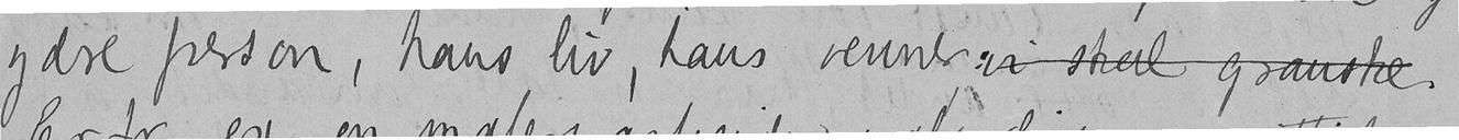ydre person, hans liv, hans venner vi skal granske.
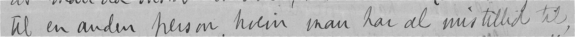til en anden person, hvem man har al mistillid til,
